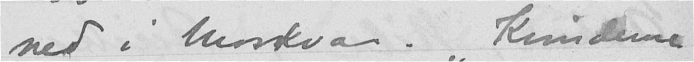ned i Moskva. "Kvinderne
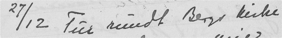27/12 Tur rundt Bergs kirke
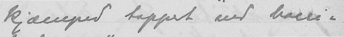kjæmped tappert ved barri-
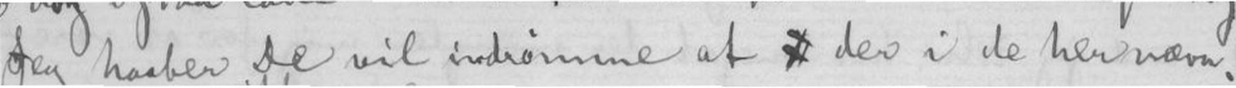Jeg haaber De vil indrømme at der i de hernævn-
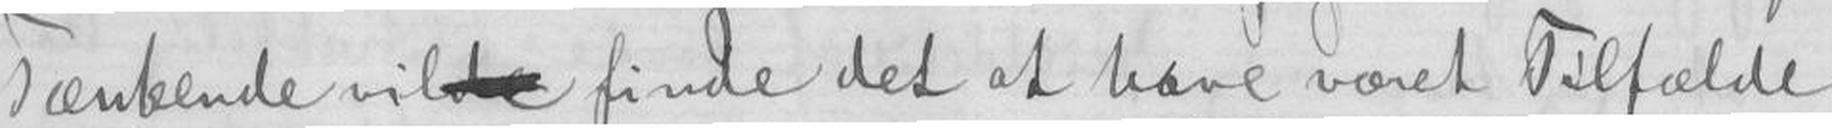Tænkende vil finde det at have været Tilfælde
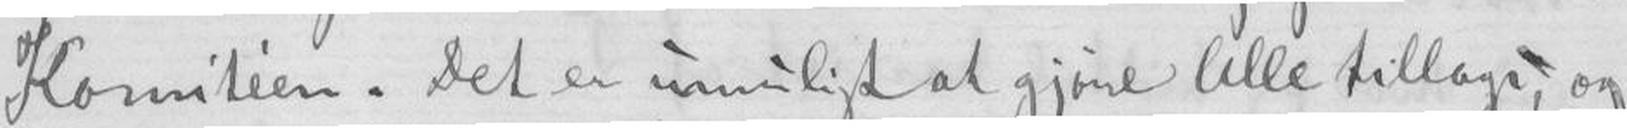Komièen. Det er umuligt at gjøre Alle tillags, og
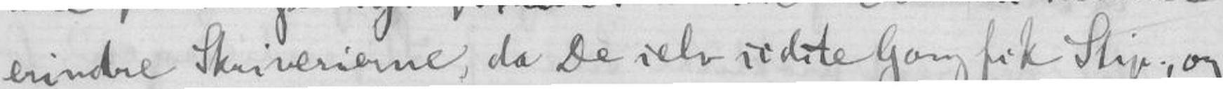erindre Skriverierne, da De selv sidste Gang fik Stip, og
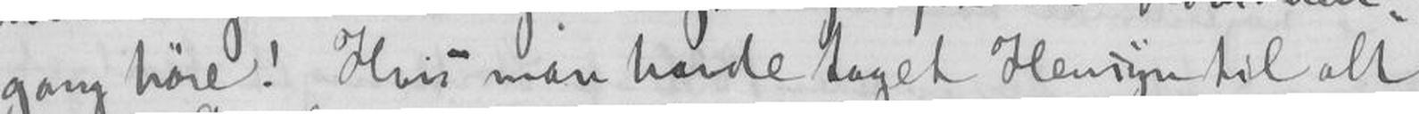gang høre! Hvis man havde taget Hensyn til alt
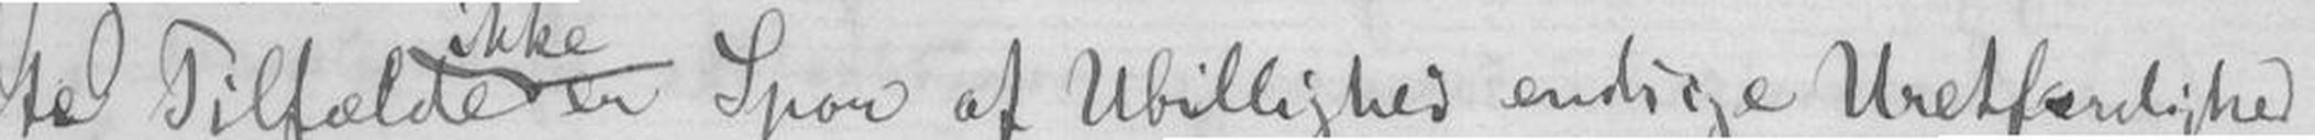te Tilfælde er Spor af Ubillighed endsige Uretfærdighed
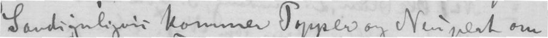Sandsynligvis kommer Popper og Neupert om
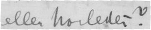eller hvorledes?
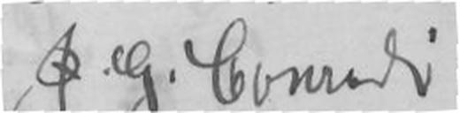J.G. Conradi
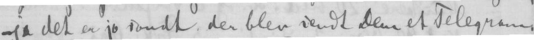ja det er jo sandt der blev sendt Dem et Telegram.
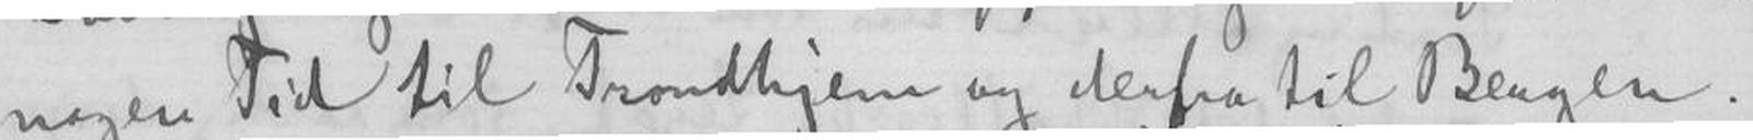nogen Tid til Tromdhjem og derfra til Bergen.
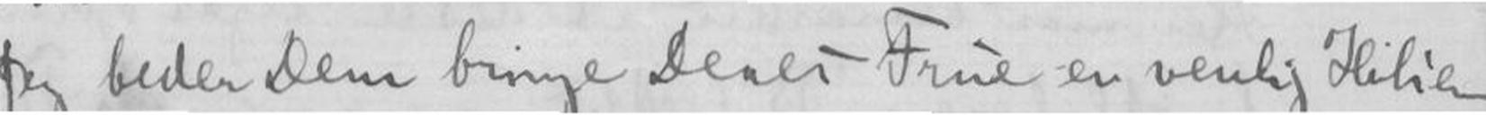Jeg beder Dem bringe Deres Frue en venlig Hilsen
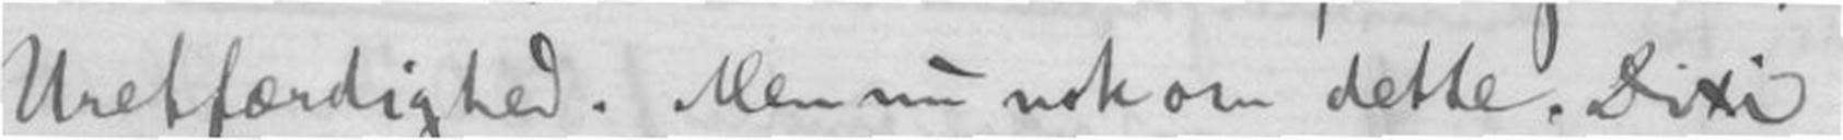Uretfærdighed. Men nu nok om dette. Dixi
